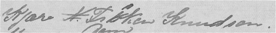Kjære N Frøken Knudsen.
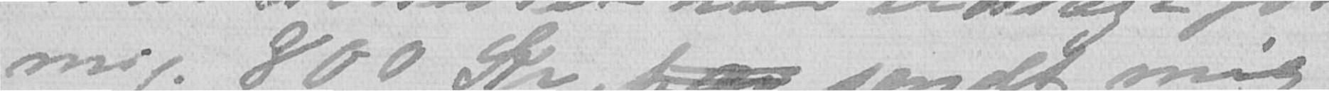mig. 800 Kr, har sendt mig
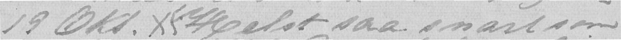19 Okt. X (Helst saa snart som
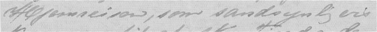Hjemreisen, som sandsynligvis
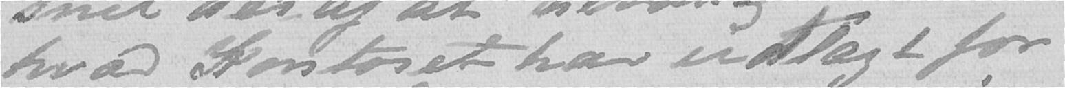hvad Kontoret har udlagt for
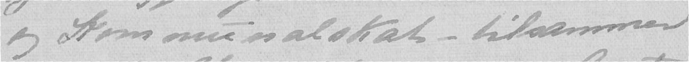og Kommunalskat - tilsammen
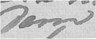dem
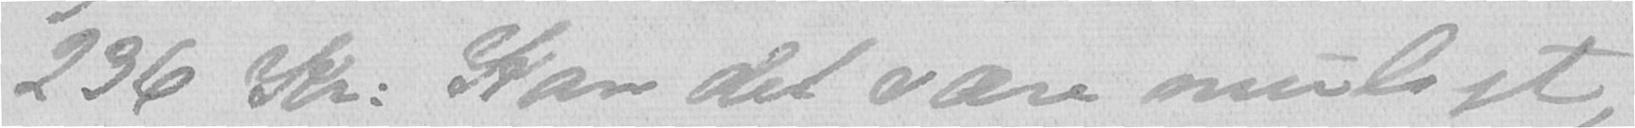236 Kr: Kan det være muligt,
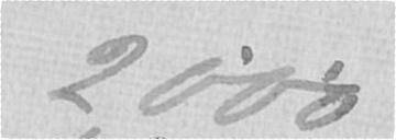2000
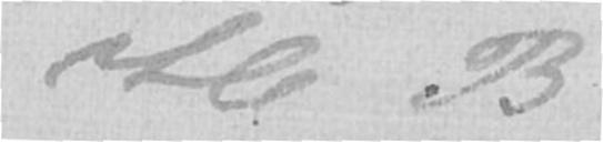H B
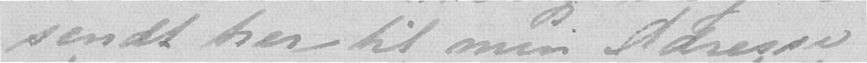sendt her til min Adresse
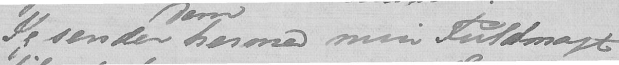Jeg sender hermed min Fuldmagt
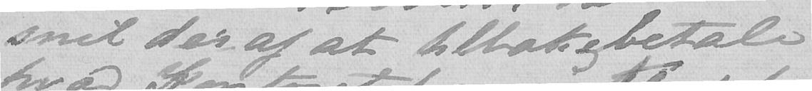snil deraf at tilbakebetale
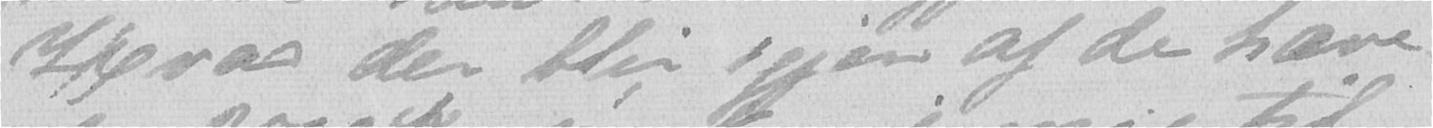Hvad der blir igjen af de hæve-
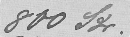800 Kr.
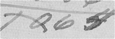1064
